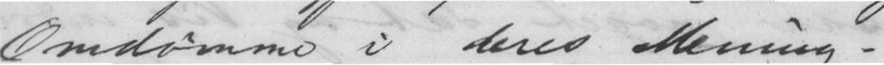Omdømme i deres Mening.
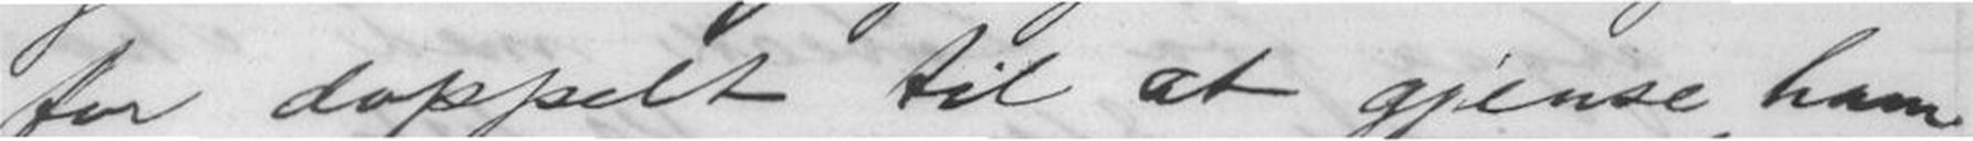for doppelt til at gjense ham
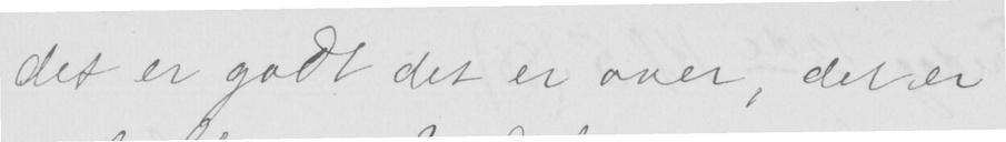det er godt det er over, det er
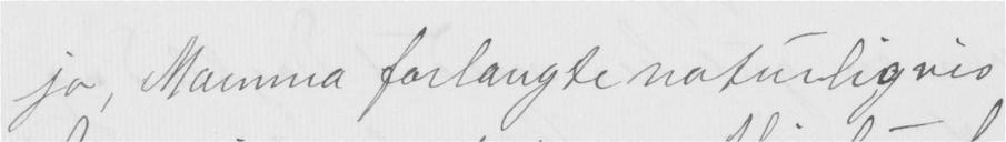jo, Mamma forlangte naturligvis
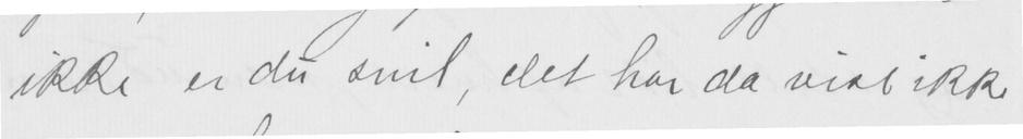ikke er du snil, det har da vist ikke
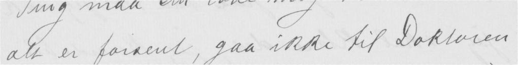alt er forsent, gaa ikke til Doktoren
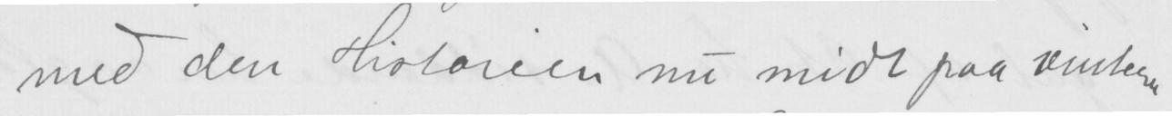med den Historien nu midt paa vinteren
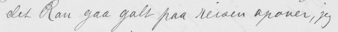det kan gaa galt paa reisen opover, jeg
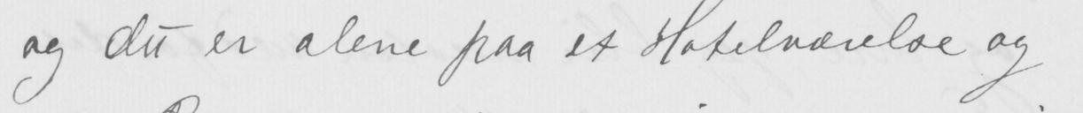og du er alene paa et Hotelværelse og
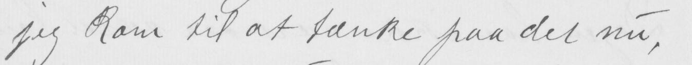jeg kom til at tænke paa det nu,
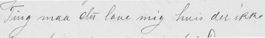Ting maa du love mig hvis det ikke
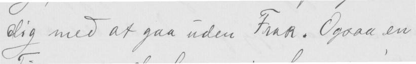dig med at gaa uden Frak. Ogsaa en
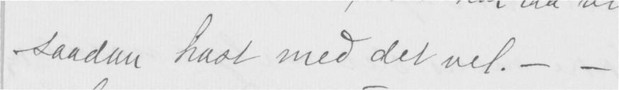saadan hast med det vel. – –
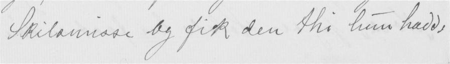Skilsmisse og fik den thi hun hadde
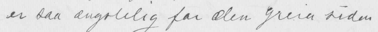er saa ængstelig for den greia siden
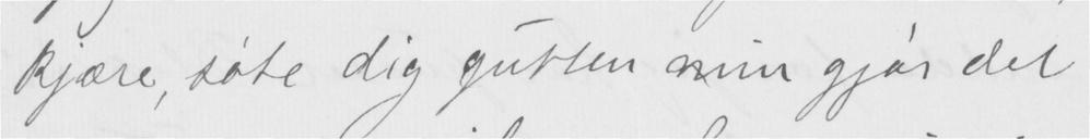kjære, søte dig gutten min gjør det
